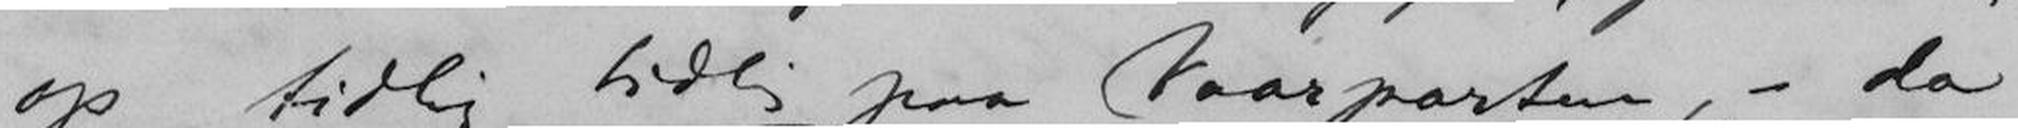op tidlig tidlig paa Vaarparten, - da
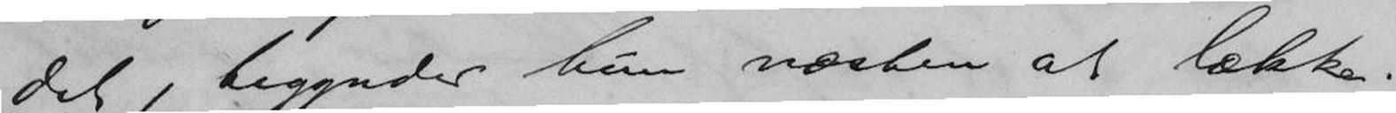det, begynder hun næsten at lække.
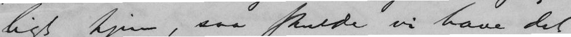ligt hjem, saa skulde vi have det
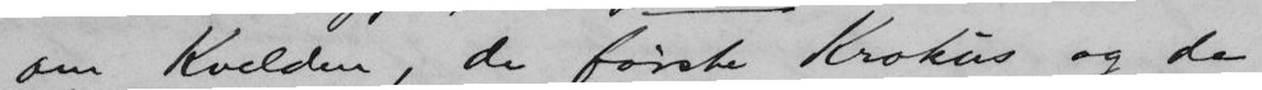om Kvelden, de første Krokus og de
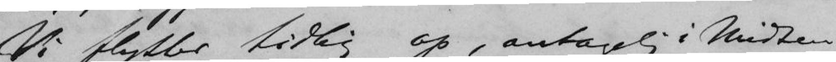Vi flytter tidlig op, antagelig i Midten
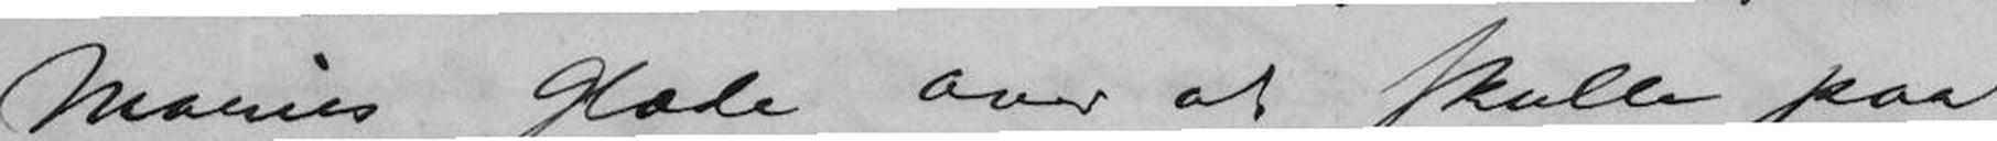Maries Glæde over at skulle paa
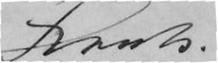Frants.
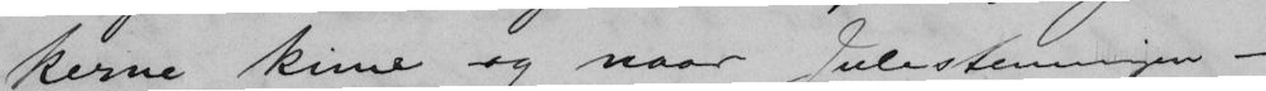kerne kime og naar Julestemningen -
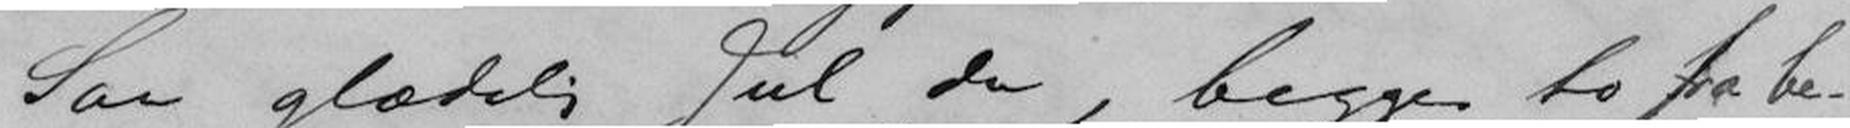Saa glædelig Jul da, begge to fra be-
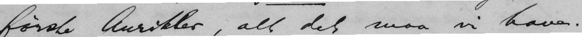første Aurikler, alt det maa vi have.
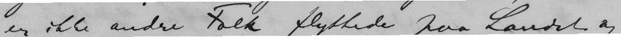er ikke andre Folk flyttede paa Landet og
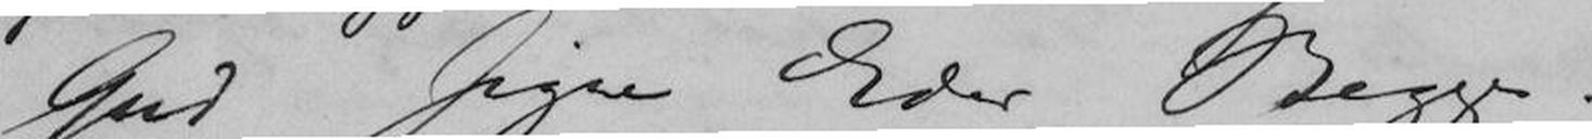Gud signe Eder Begge!
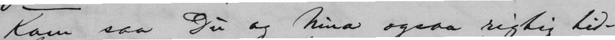Kom saa Du og Nina ogsaa rigtig tid-
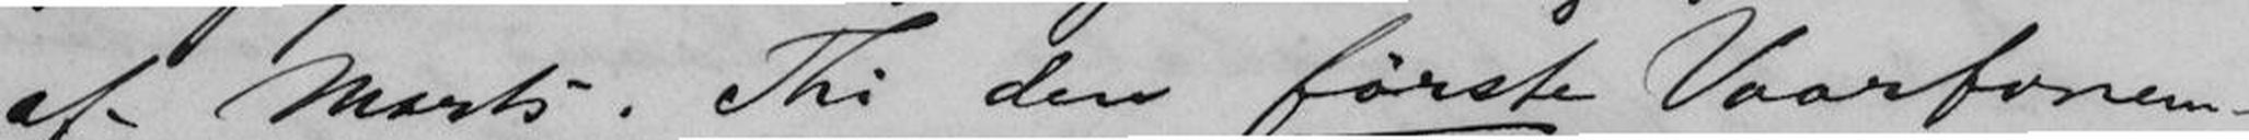af Marts. Thi den første Vaarfornem-
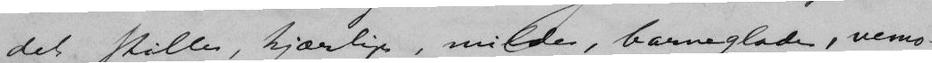det stille, kjærlige, milde, barneglade, vemo-
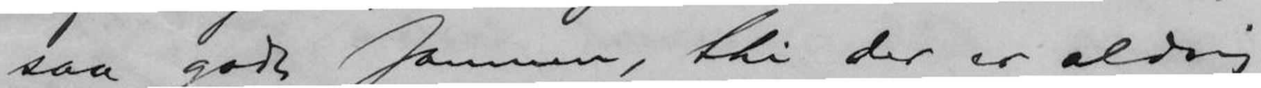saa godt sammen, thi der er aldrig
